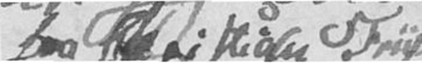fra  frue Friis
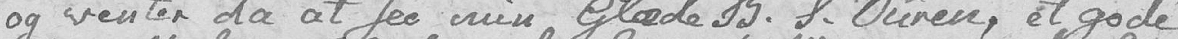og venter da at see min Glæde B. S. Ouren, et gode
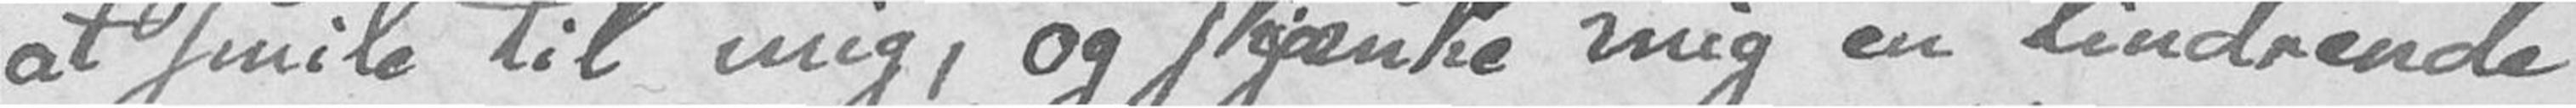at smile til mig, og skjænke mig en lindrende
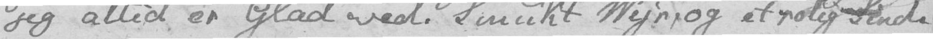jeg altid er Glad ved. Smukt Vejr, og et rolig Sind.
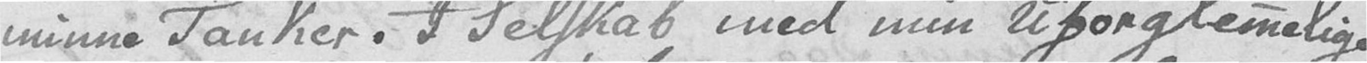minne Tanker. I Selskab med min uforglemmelige
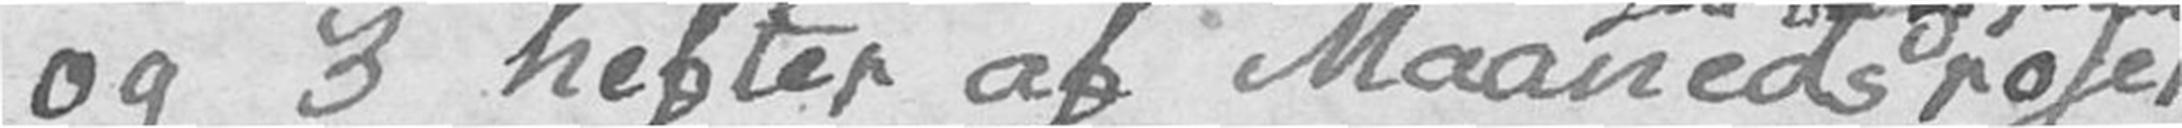og 3 hefter af Maanedsroser
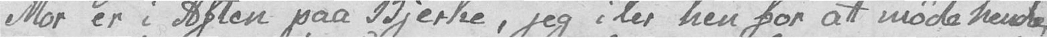Mor er i Aften paa Bjerke, jeg iler hen for at møde hende
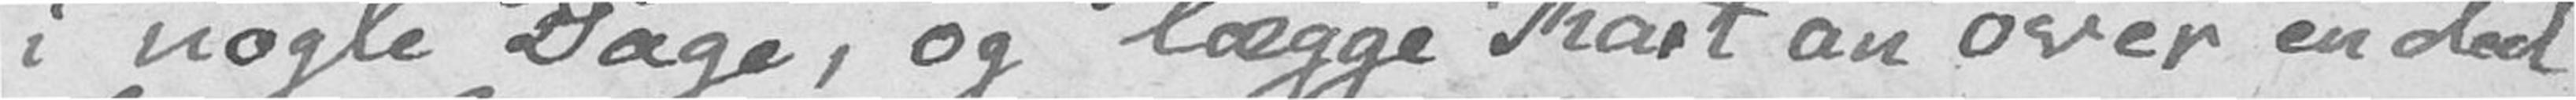i nogle Dage, og lægge Kart an over en deel
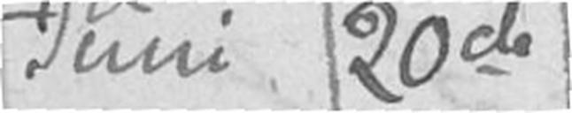Juni 20de
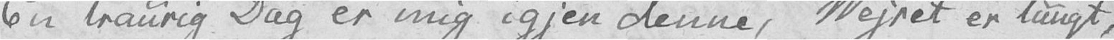En traurig Dag er mig igjen denne, Vejret er tungt,
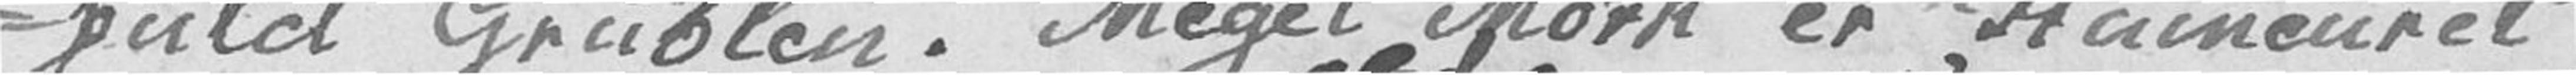-fuld Grublen. Meget Mørk er Humeuret
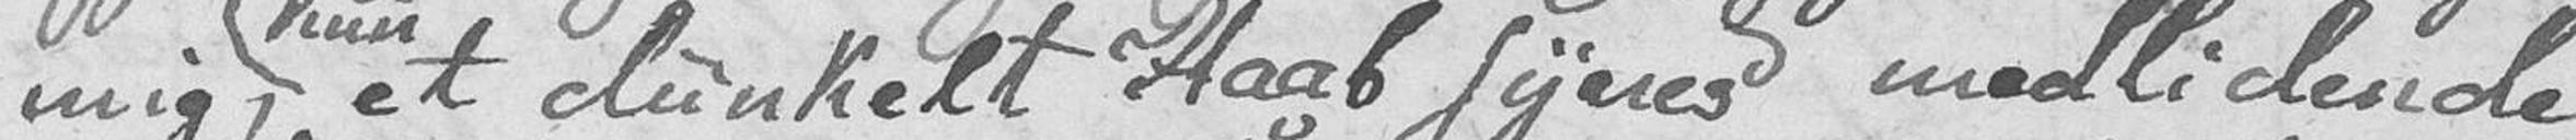mig, kun et dunkelt Haab synes medlidende
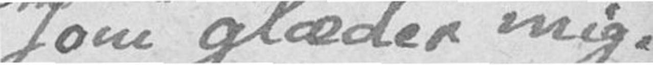som glæder mig.
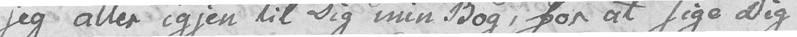jeg atter igjen til Dig min Bog, for at sige Dig
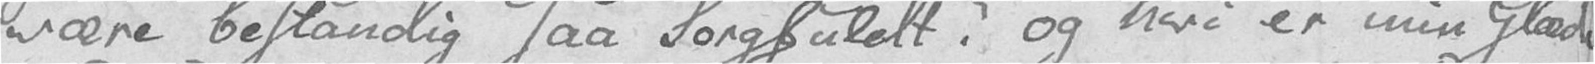være bestandig saa Sorgfuldt? og hvi er min Glæde
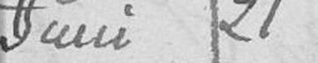Juni 21
